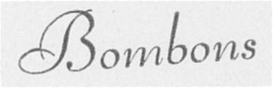Bombons
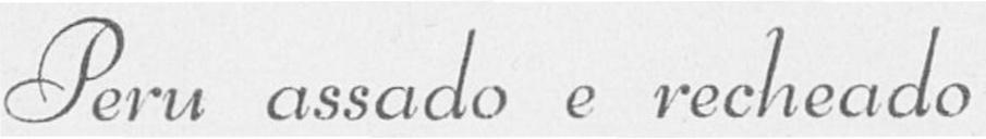Peru assado e recheado
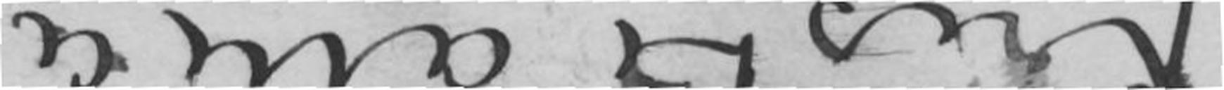Kristiania
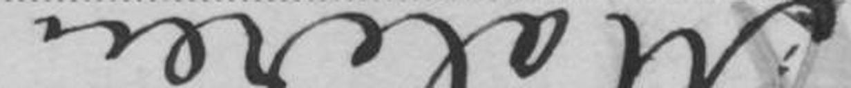Maleren
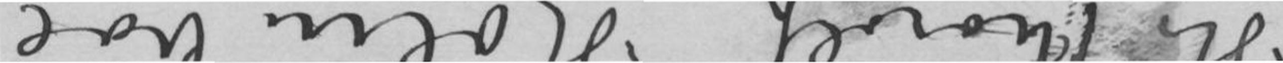Hr Thorolf Holmboe
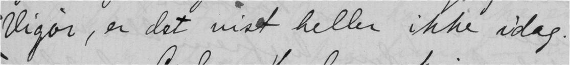Vigør, er det vist heller ikke idag.
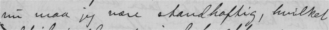nu maa jeg være standhaftig, hvilket
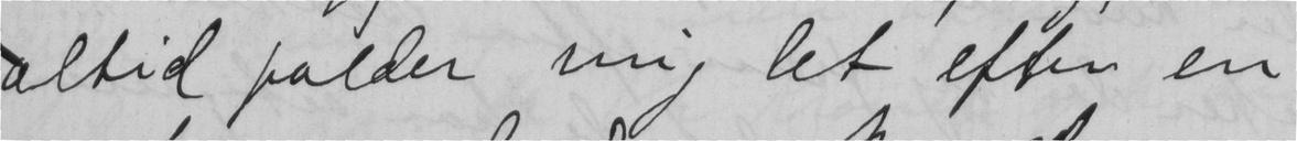altid falder mig let efter en
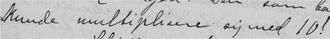kunde miltiplisere sig med 10!
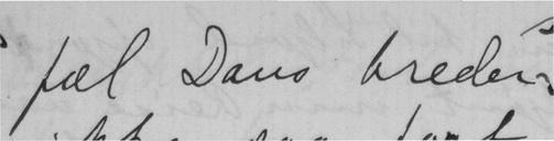fæl Dans breder
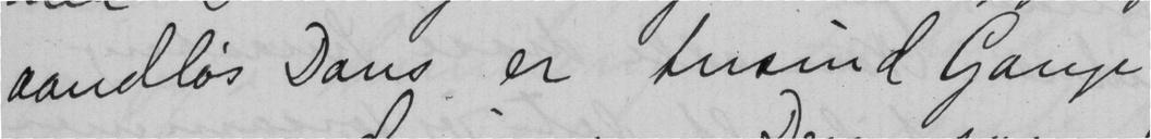aandløs Dans er tusind Gange
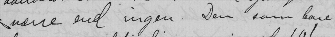værre end ingen. Den som bare
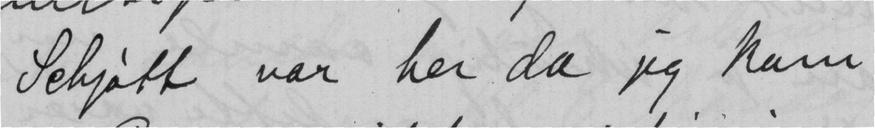Schjøtt var her da jeg kom
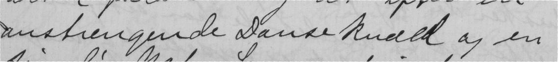anstrengende Dansekvæld og en
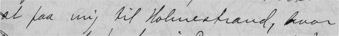at faa mig til Holmestrand, hvor
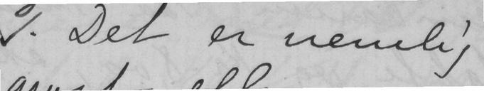. Det er nemlig
